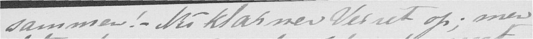sammen. Nu klarner Veiret op; men
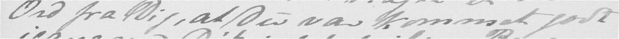Ord fra Dig, at Du var kommet godt
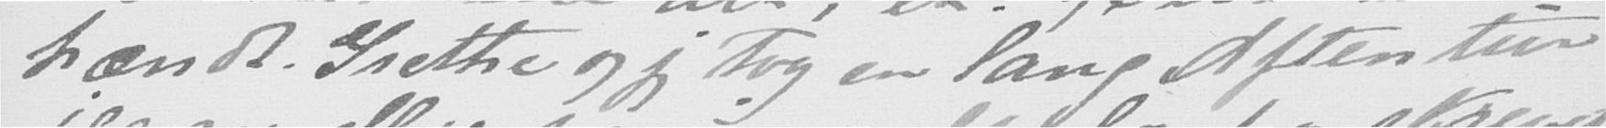hændt. Grethe og jeg tog en lang Aftentur
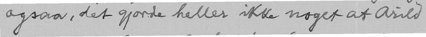ogsaa, det gjorde heller ikke noget at Arild
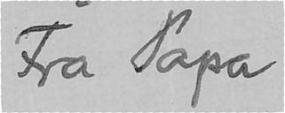Fra Papa
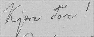Kjære Tore!
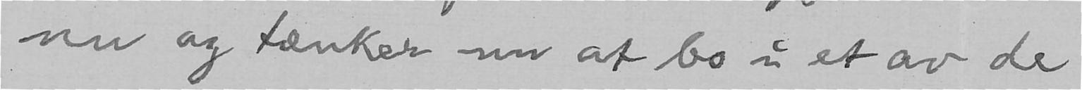nu og tænker nu at bo i et av de
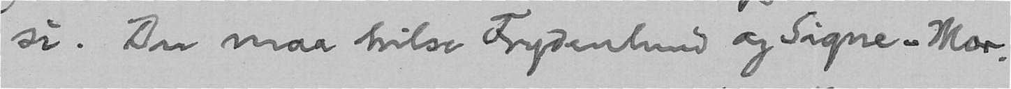si. Du maa hilse Frydenlund og Signe-Mor.
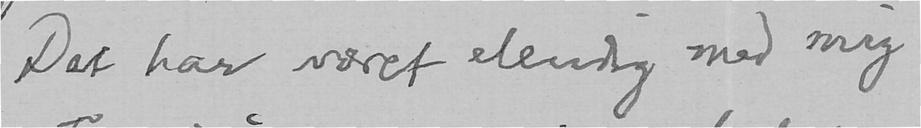Det har været elendig med mig
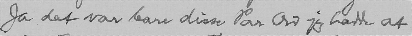Ja det var bare disse Par Ord jeg hadde at
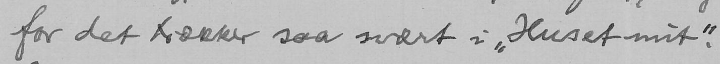for det trækker saa svært i "Huset mit".
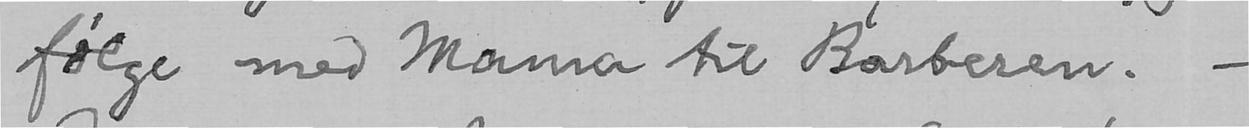følge med Mama til Barberen. -
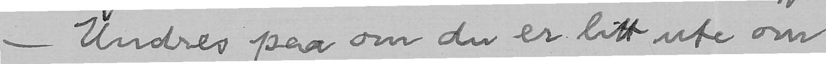- Undres paa om du er litt ute om
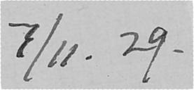7/11. 29.
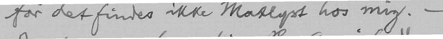for det findes ikke Matlyst hos mig. -
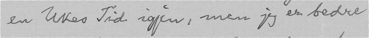en Ukes Tid igjen, men jeg er bedre
